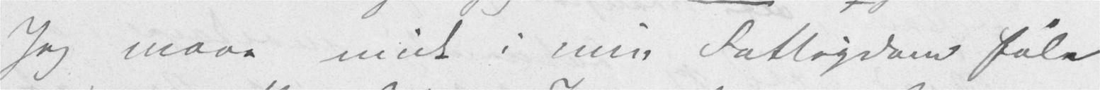Jeg maae midt i min Fattigdom føle
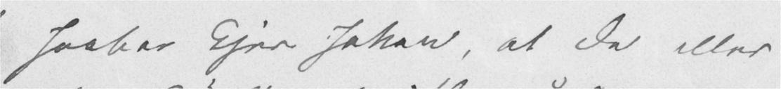Jeg haaber kjere Johan, at De eller
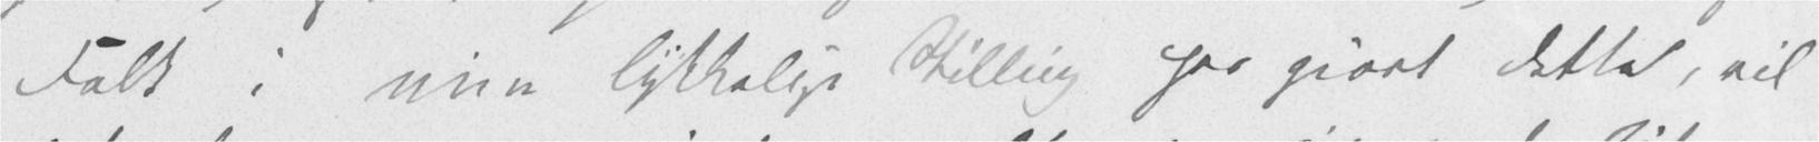Folk i min lykkelige Stilling har gjort dette, vil
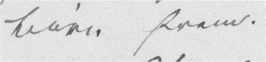Børn frem.
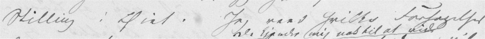Stilling i Øiet. Jeg veed hvilke Forsagelser
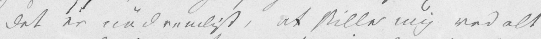det er nødvendigt, at skille mig ved alt
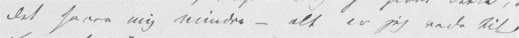det saare mig mindre – alt er jeg rede til
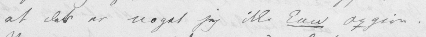at det er noget jeg ikke kan opgive.
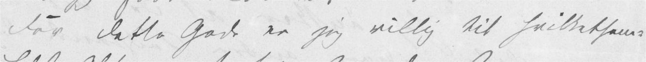For dette Gode er jeg villig til hvilketsom-
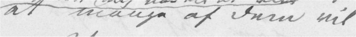at mange af dem vil
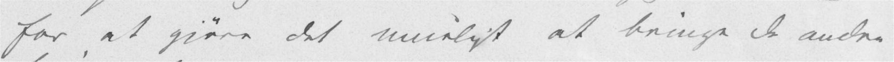for at gjøre det mueligt at bringe de andre
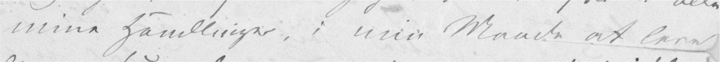mine Handlinger, i min Maade at leve
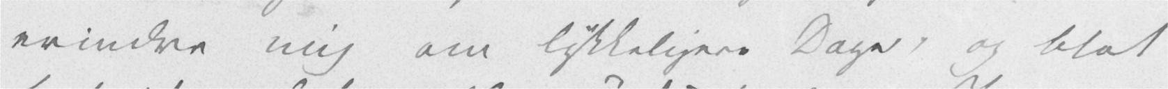erindre mig om lykkeligere Dage, og blot
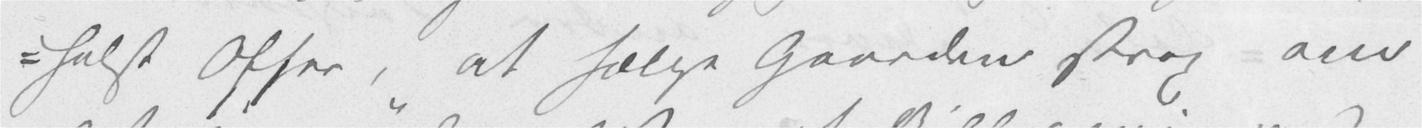helst Offer, at sælge Gaarden strax om
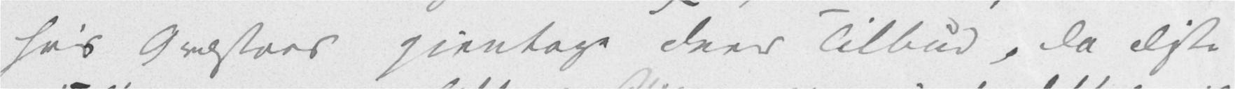hvis Qvæstors gjentage deres Tilbud, da disse
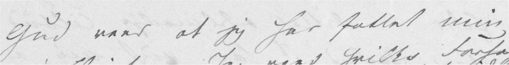Gud veed at jeg har fattet min
